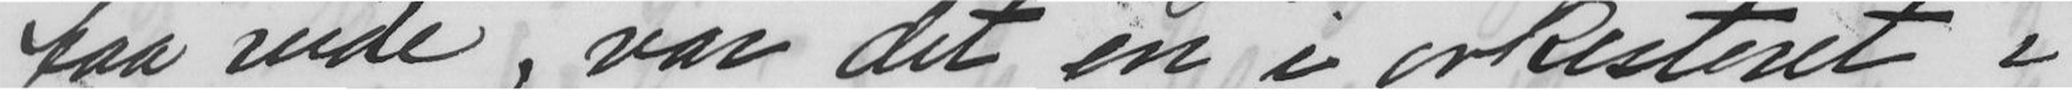faa vide, var det en i orkesteret i
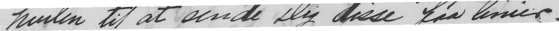hvilen til at sende Dig disse faa linier.
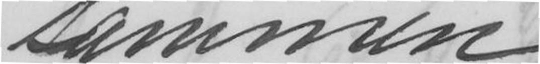sammen
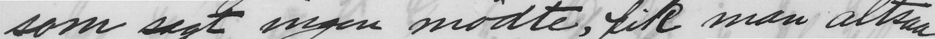som sagt ingen mødte, fik man altsaa
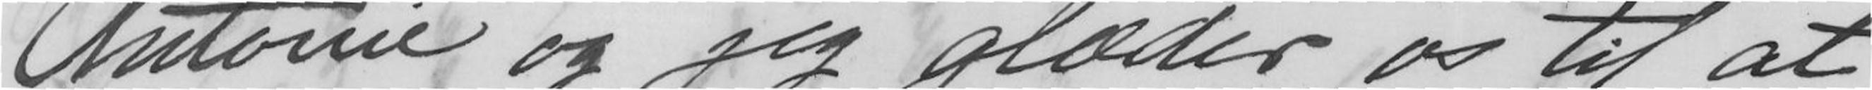Antonie og jeg glæder os til at
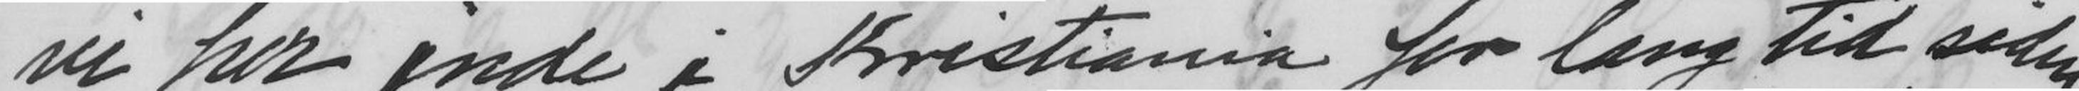vi her inde i Kristiania for lang tid siden
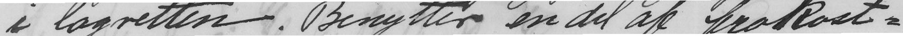i lagretten. Benytter en del af frokost=
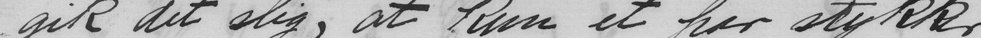gik det slig, at kun et par stykker
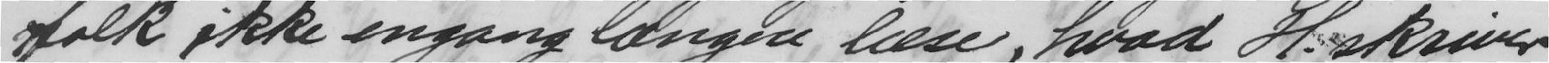folk ikke engang længere læse, hvad H. skriver.
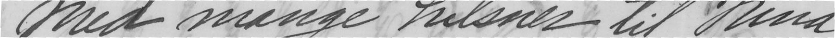Med mange hilner til Nina
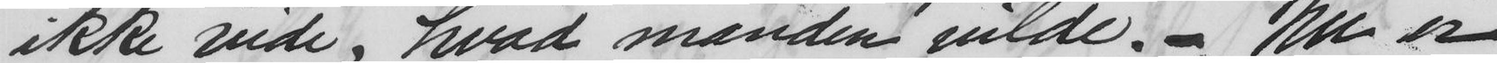ikke vide, hvad manden vilde. - Nu er
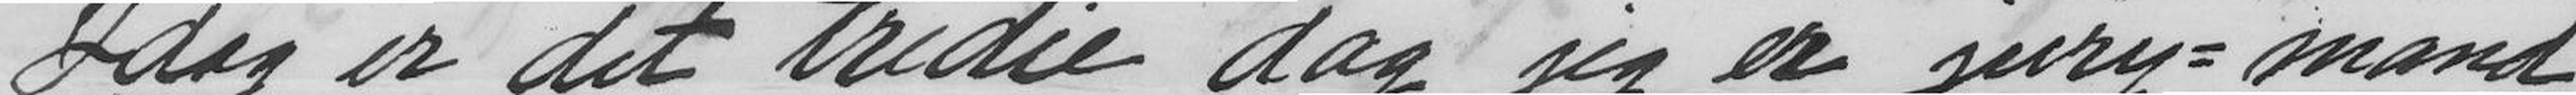Idag er det tredie dag jeg er jury=mand
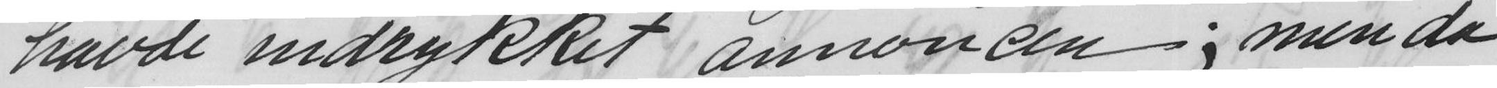havde indrykket annoncen; men da
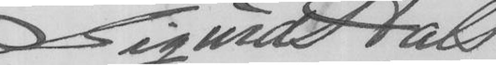Sigurd Hals.
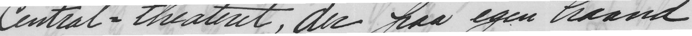Central=theatret, der paa egen haand
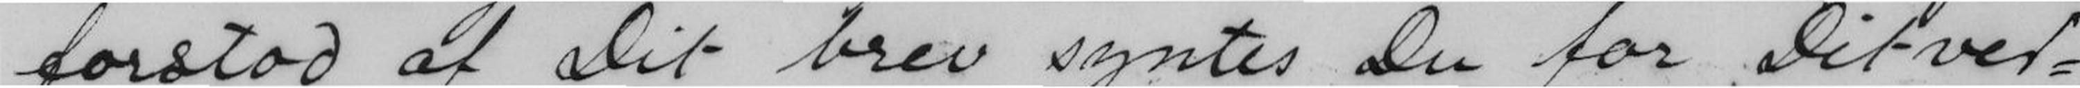forstod af Dit brev syntes Du for Dit ved-
